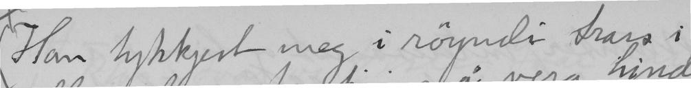Han tykkjest meg i röyndi trass i
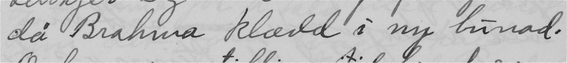då Brahma klædd i ny bunad.
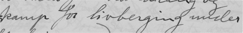kamp for livberging under
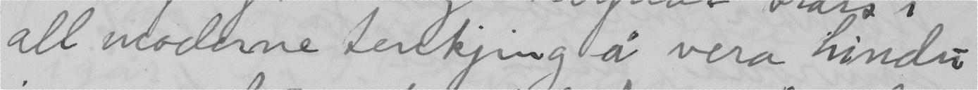all moderne tenkjing å vera hindu
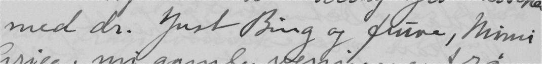med dr. Just Bing og fruve, Mimi
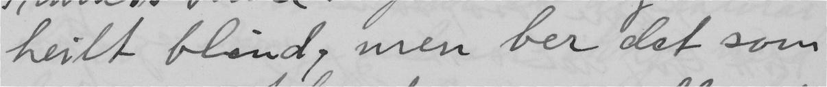heilt blind, men ber det som
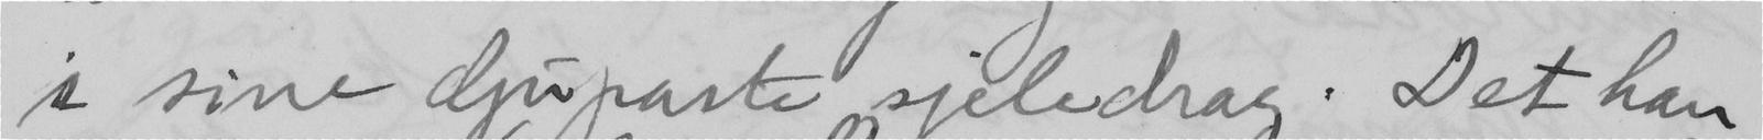i sine djupaste sjeledrag. Det han
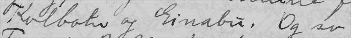Kolbotn og Einabu. Og so
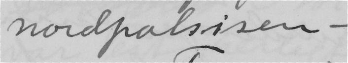nordpolsisen –
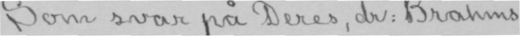Som svar på Deres, dr: Brahms
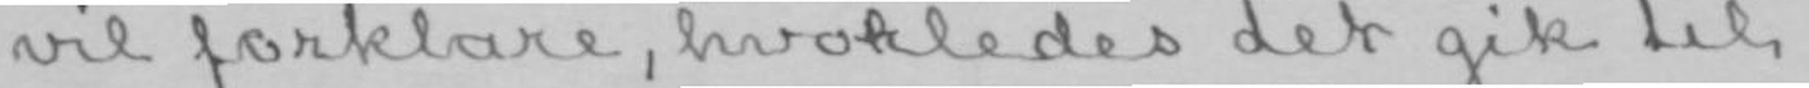vil forklare, hvorledes det gik til
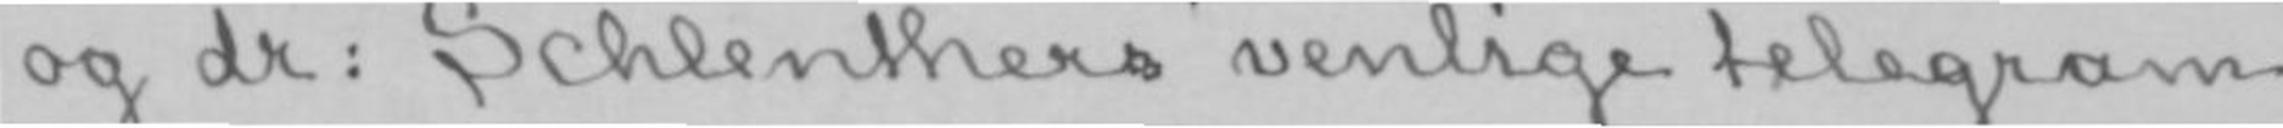og dr: Schlenthers venlige telegram
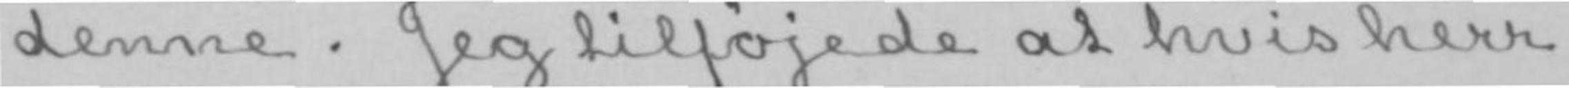denne. Jeg tilføjede at hvis herr
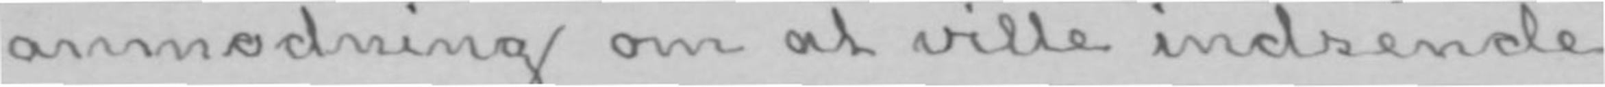anmodning om at ville indsende
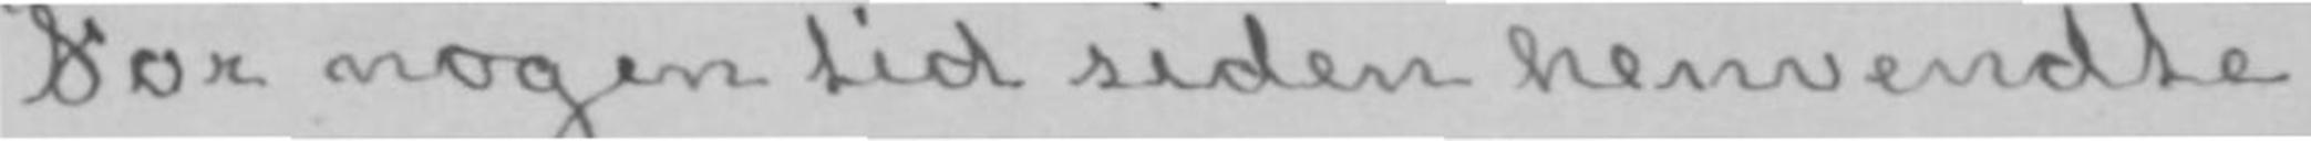For nogen tid siden henvendte
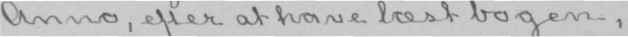Anno, efter at have læst bogen,
